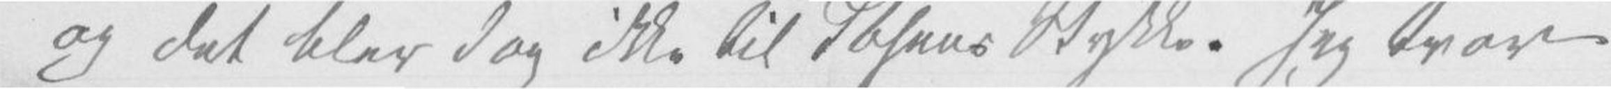og det blev dog ikke til Ibsens Stykke. Jeg tror
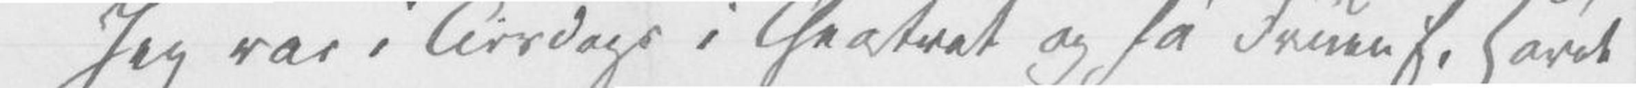Jeg var i Tirsdags i Theatret og så «Fruen f. Havet[».]
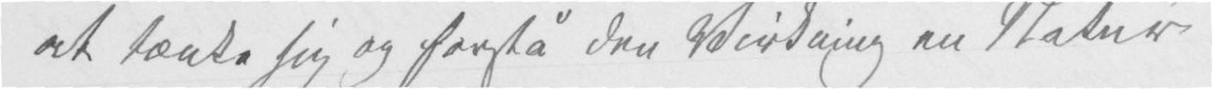at tænke sig og forstå den Virkning en Natur
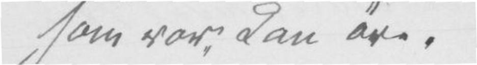som vor kan øve.
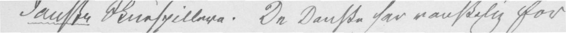danske Skuespillere. De Danske har vanskelig for
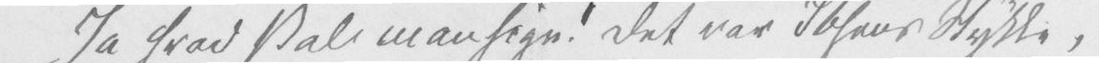Ja hvad skal man sige! Det var Ibsens Stykke,
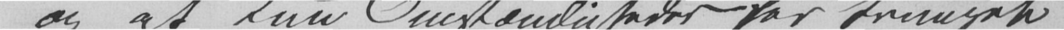og at kun Omstændigheder har tvunget
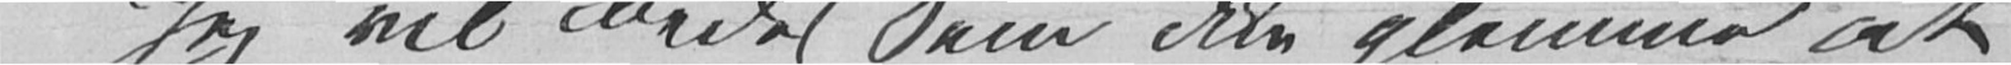Jeg vil bede Dem ikke glemme at
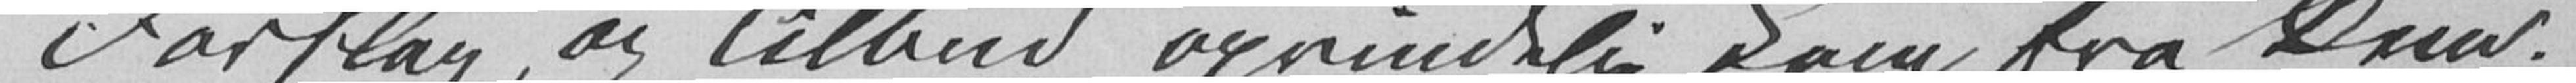Forslag og Tilbud oprindelig kom fra Dem,
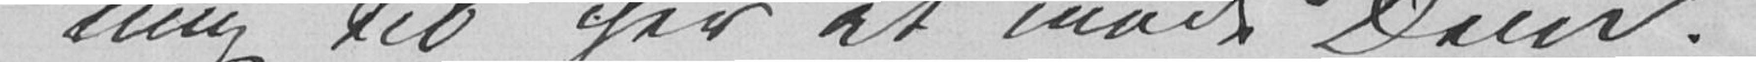mig til her at møde Dem.
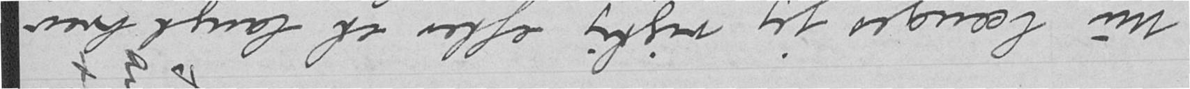nu længes jeg vigtig efter et langt brev
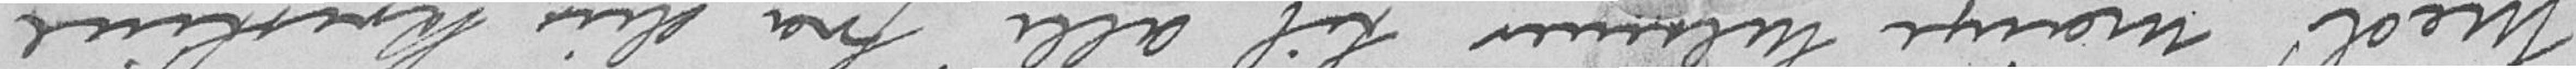Med mange hilsner til alle fra din Kristine
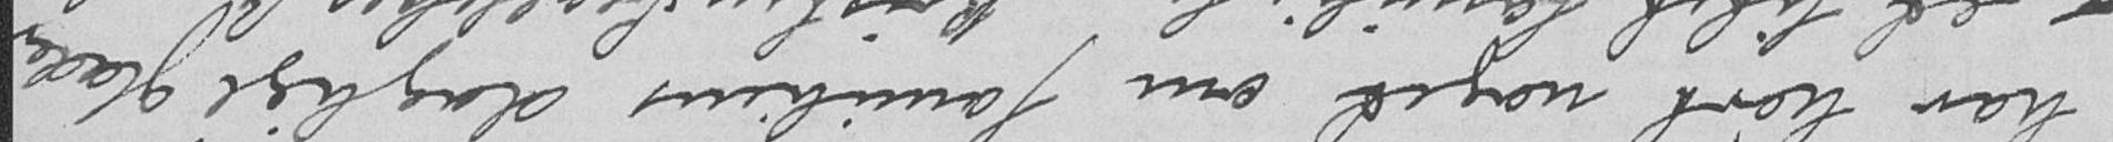har hørt noget om familiens daglige glæder
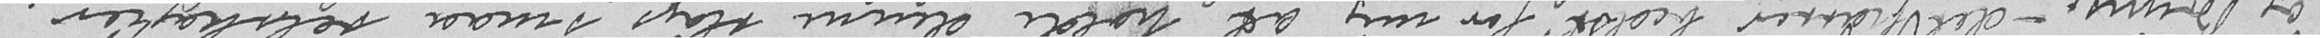og Bryns, - det passer bedst for mig at holde denne slags smaa selskaper.
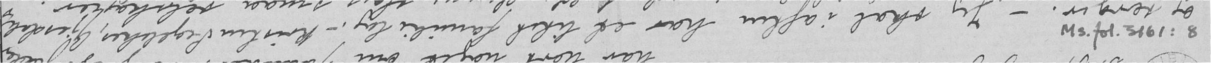og sorger. Jeg skal i aften har et litet familielag, - Kristen Segelckes, Gjesdahls
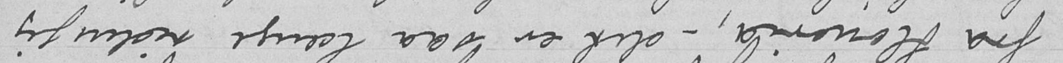fra Honoria, - det er saa længe siden jeg
